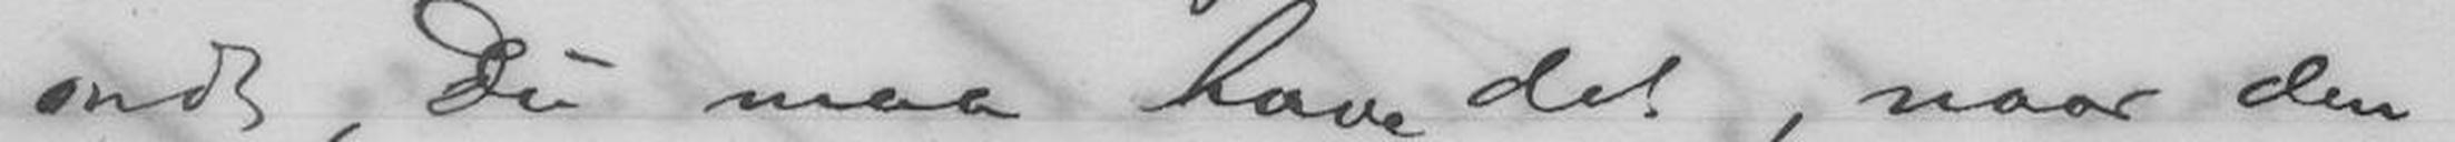ondt, Du maa have det, naar den
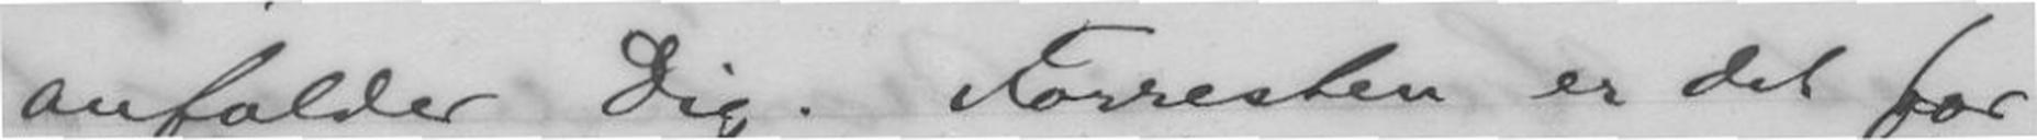anfalder Dig. Forresten er det for
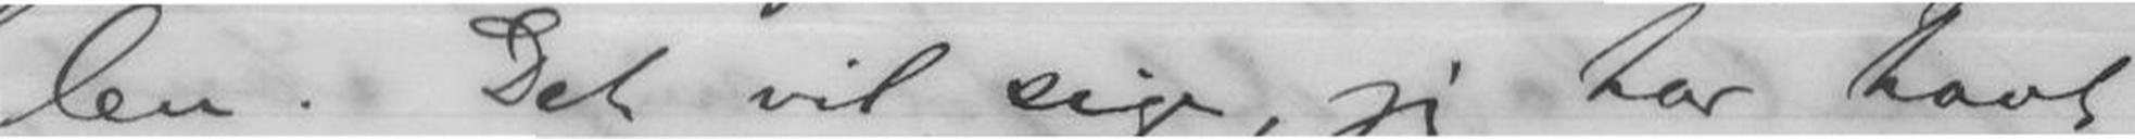len. Det vil sige, jeg har havt
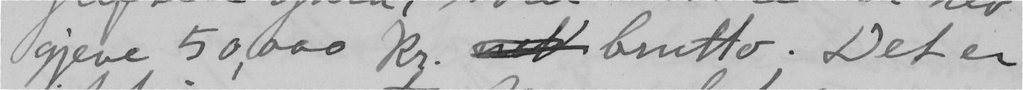gjeve 50,000 kr.  brutto. Det er
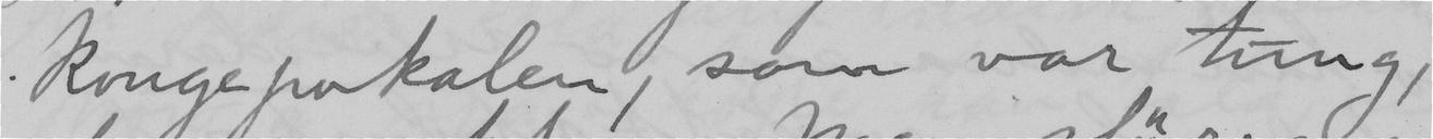Kongepokalen, som var tung,
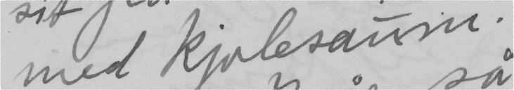med kjolesaum.
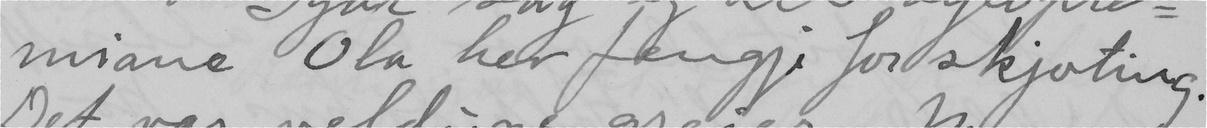Ola hev fengji for skjoting.
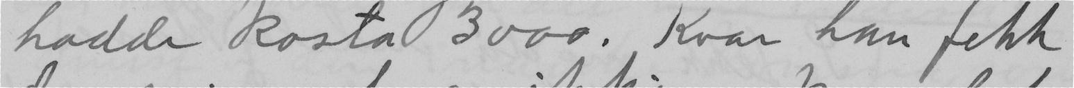hadde kosta 3000. Kvar han fekk
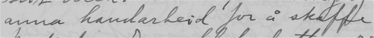anna handarbeid for å skaffe
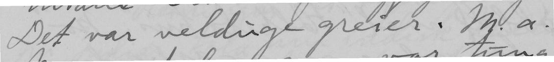Det var velduge greier. M.a.
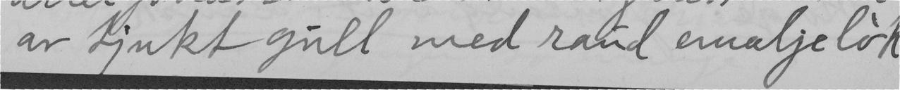av tjukt gull med raud emaljelok
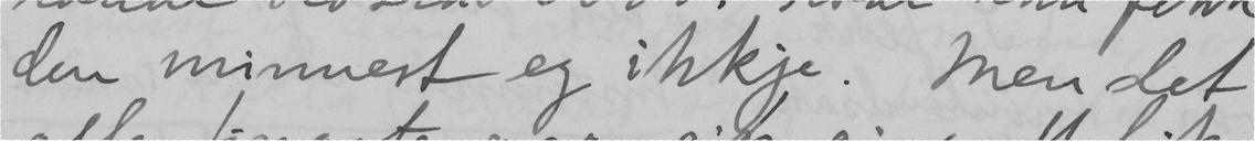den minnest eg ikkje. Men det
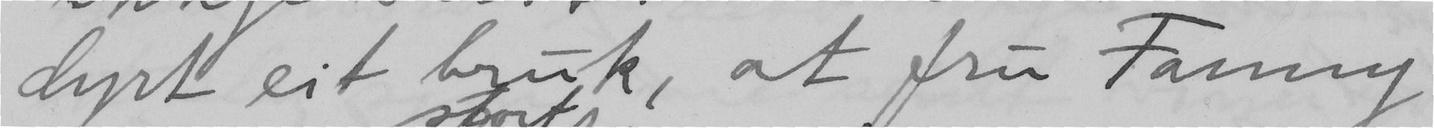dyrt eit bruk, at fru Fanny
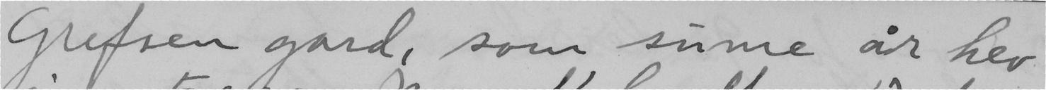Grefsen gard, som sume år hev
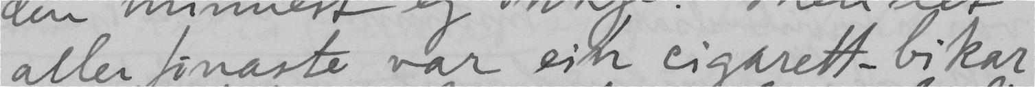aller finaste var ein cigarett-bikar
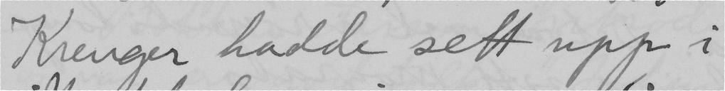Kungen hadde sett upp i
